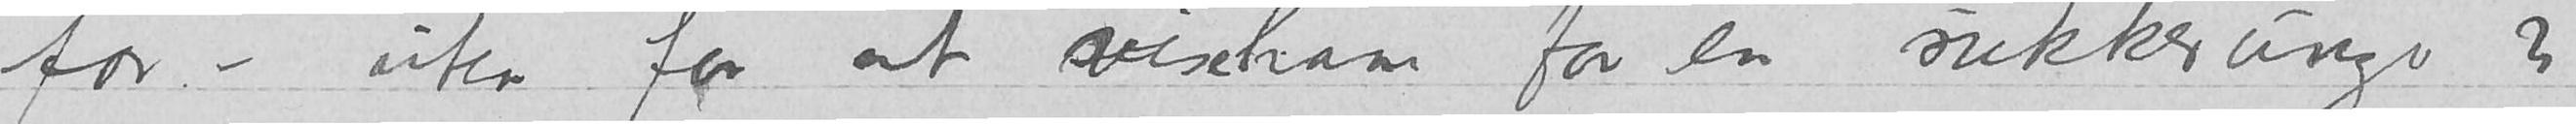far – uten for at  vise ham for en sukkerunge jeg
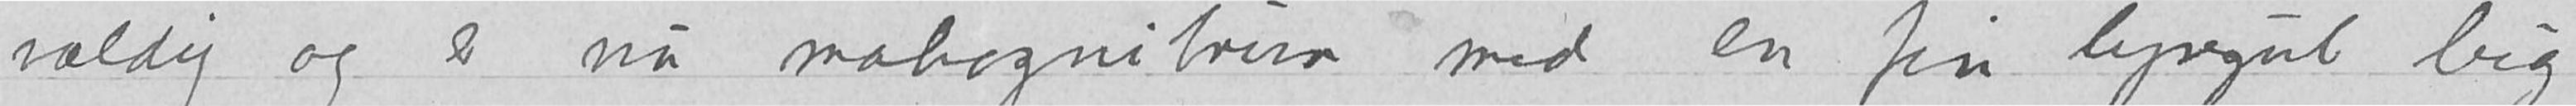vældig og er nu mahognibrun med en fin lysegul lug
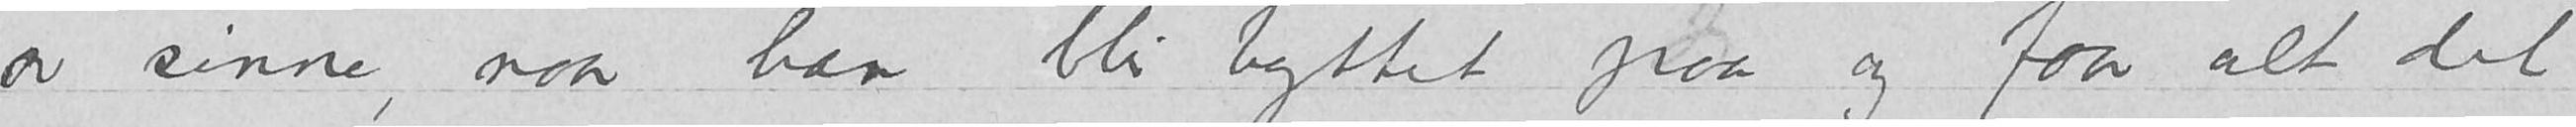av sinne, naar han blir byttet paa og faar alt det
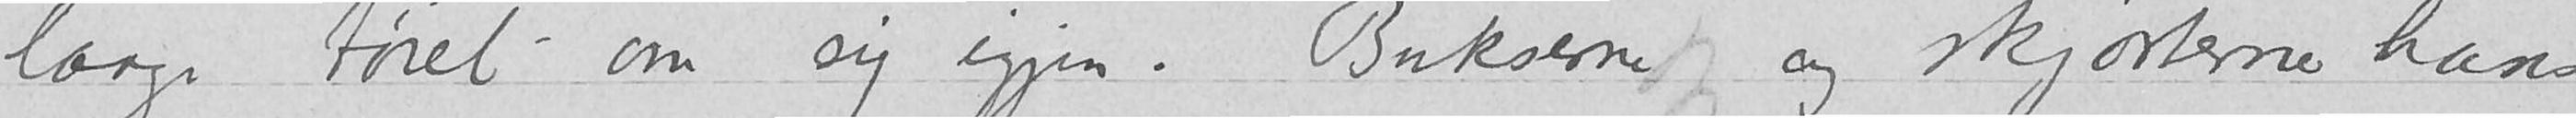lange tøiet om sig igjen. Bukserne og skjorterne hans
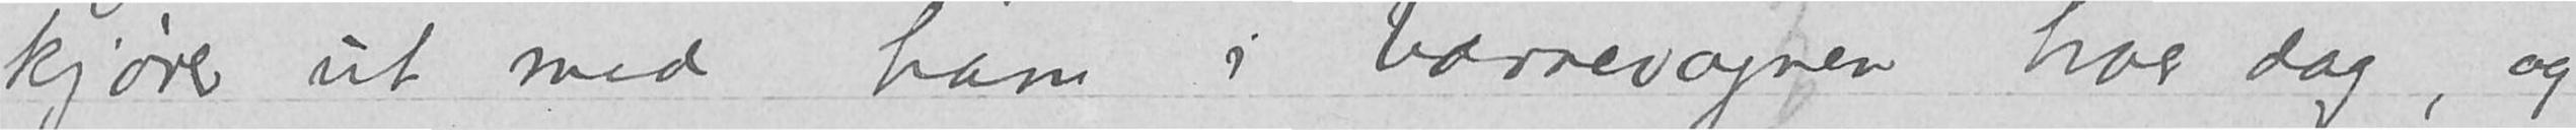kjører ut med ham i barnevognen hver dag, og
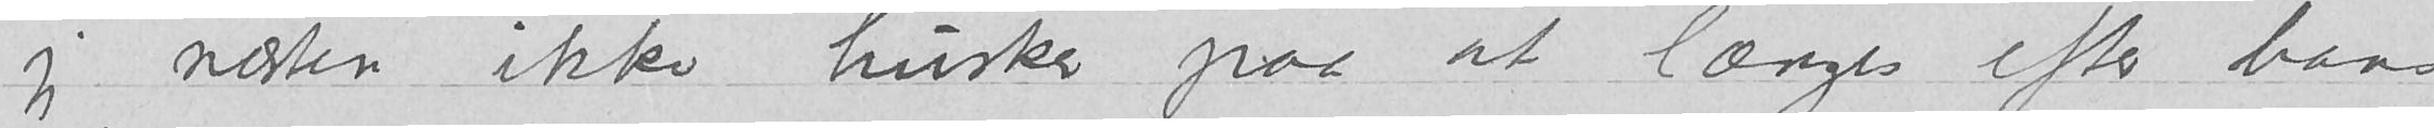jeg næsten ikke husker paa at længes efter hans
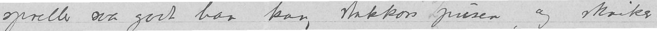spreller saa godt han kan, stakkars pusen, og skriker
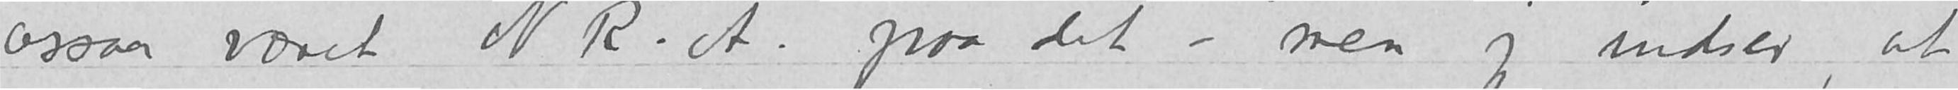ogsaa været NR.A. paa det – men jeg indset, at
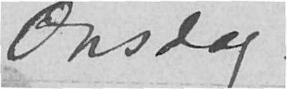Onsdag
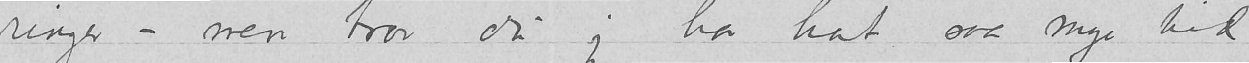ringer – men tror du jeg har hat saa mye tid
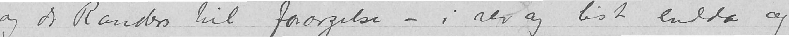og dr. Randers til forargelse – i rev og list endda og
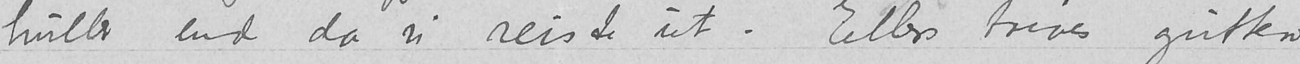huller end da vi reiste ut. Ellers trives gutten
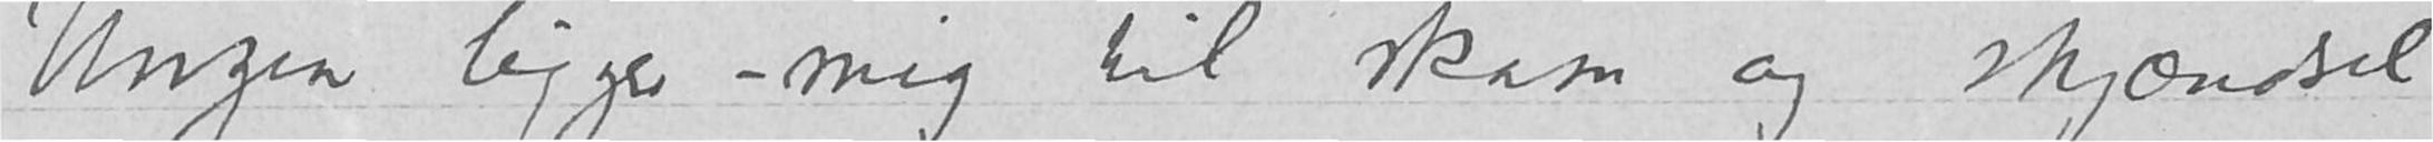Ungen ligger – mig til skam og skjændsel
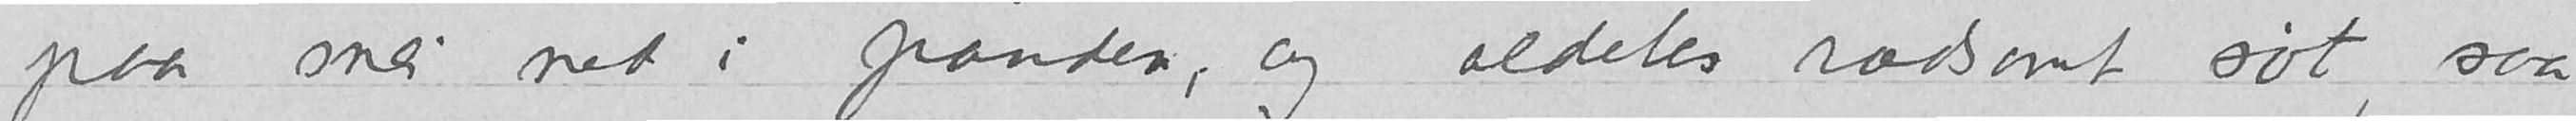paa snei ned i panden, og aldeles rædsomt søt, saa
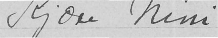Kjære Nini,
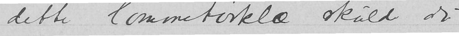Dette lommetørklæ skulde du
